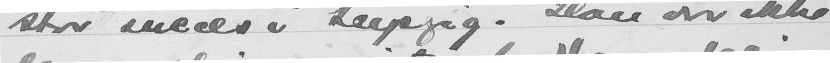stor succes i Leipsig. Han var ikke
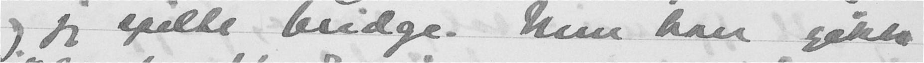og jeg spilte bridge. Men han gikk
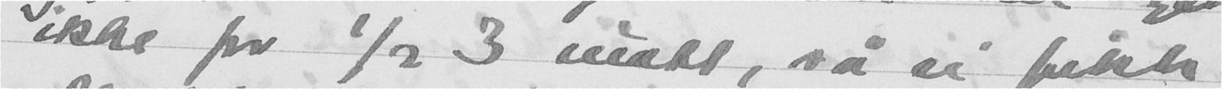ikke for 1/2 3 inatt, så vi fikk
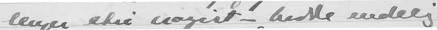lenger stri nazist - hadde endelig
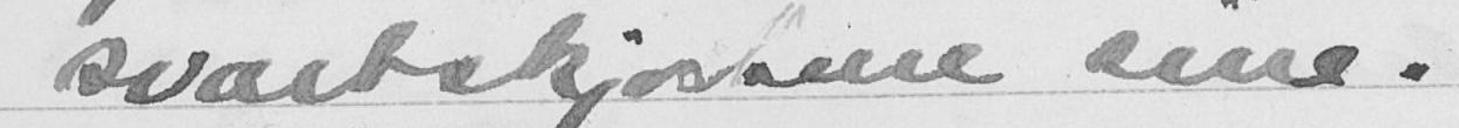svartskjortene sine.
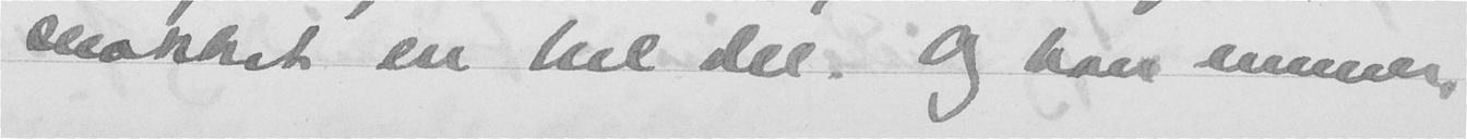snakket en hel del. Og han mener,
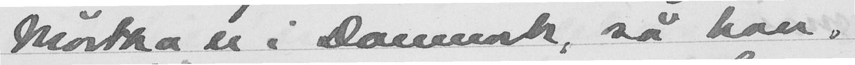Märtha er i Danmark, sa han,
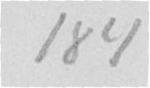184
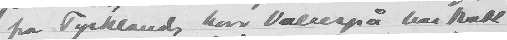fra Tyskland hvor Valuesjiå har hatt
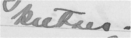kretsen.
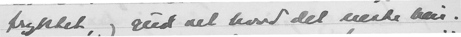fryktet, og gud vet hvad det neste blir.
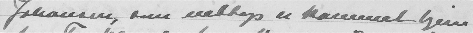Johansen, som nettop er kommet hjem
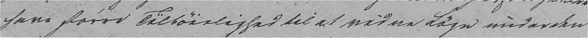have større Tilbøielighed til at vidne Løgn under den
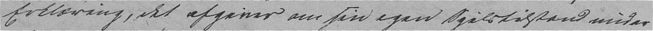Erklæring, det afgiver om sin egen Sjelstilstand under
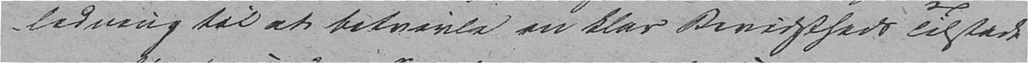ledning til at betvivle en klar Bevidstheds Tilstede-
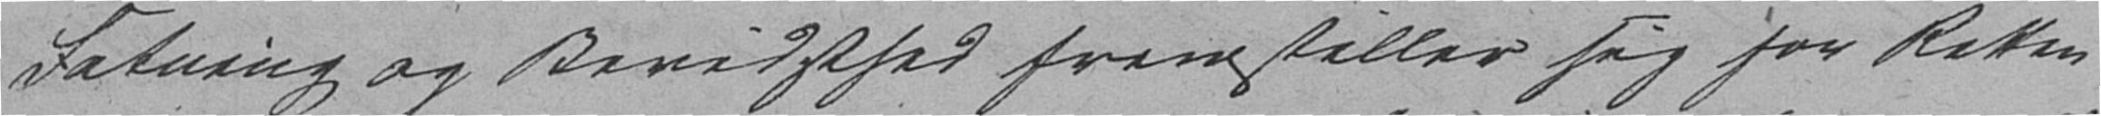Fatning og Bevidsthed fremstiller sig for Retten
